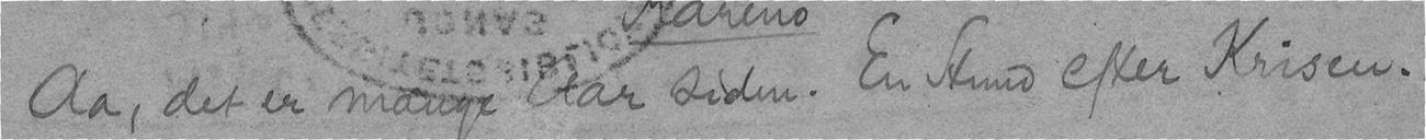Aa, det er mange Aar siden. En Stund efter Krisen.
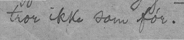tror ikke som før.
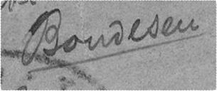Bondesen
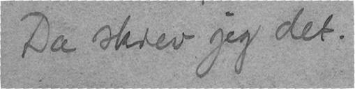Da skrev jeg det.
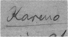Kareno
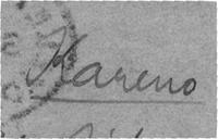Kareno
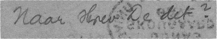Naar skrev De det?
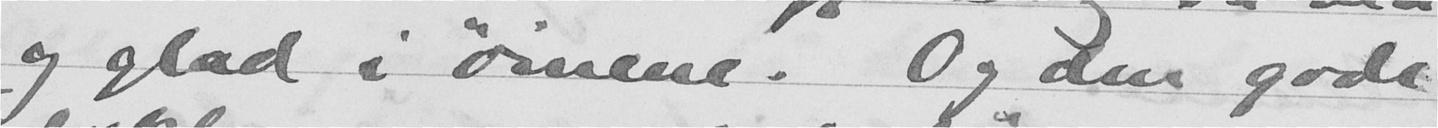og glad i øinene. Og den gode
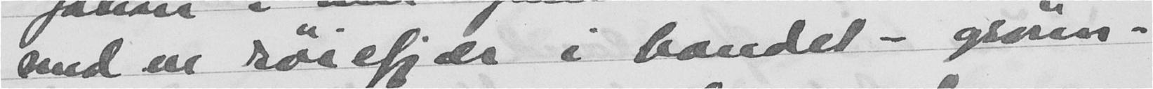med en røiefjær i bandet - grønn-
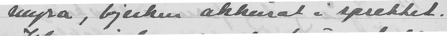myra, bjerken akkurat i sprettet.
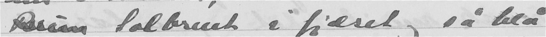Solbrent i fjæset og så blå
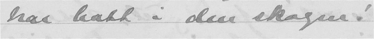har hatt i den skogen!
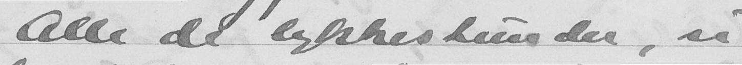Alle de lykkestunder, vi
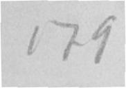179
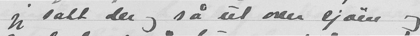jeg satt der og så ut over sjøen og
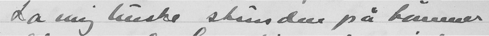La mig huske stunden på bommer
